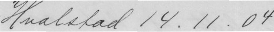Hvalstad 14.11.04
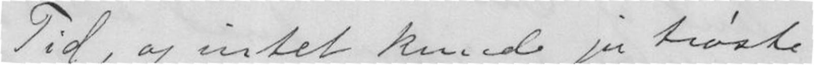Tid, og intet kunde jo trøste
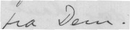fra Dem.
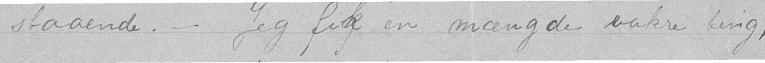staaende. – Jeg fik en mængde vakre ting,
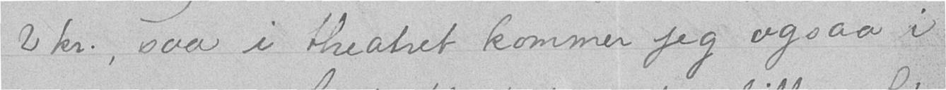2kr, saa i theatret kommer jeg ogsaa i
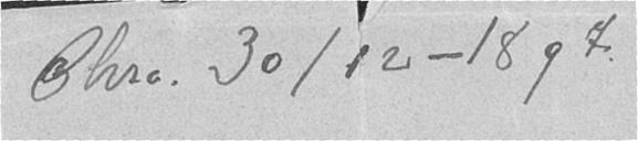Chra. 30/12-1897.
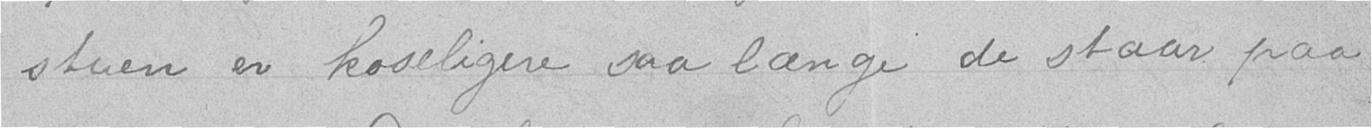stuen er koseligere saa længe de staar paa
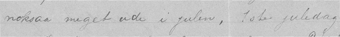noksaa meget ude i julen. 1ste juledag
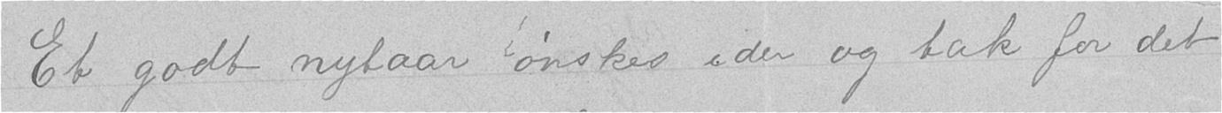Et godt nytaar ønskes eder og tak for det
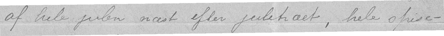af hele julen næst efter juletræet, hele spise-
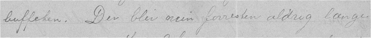buffeten. Der blir nuu forresten aldrig længe
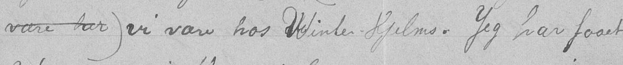være her) vi være hos Winter-Hjelms. Jeg har faaet
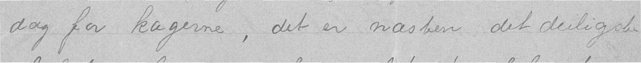dog for kagerne; det er næsten det deiligste
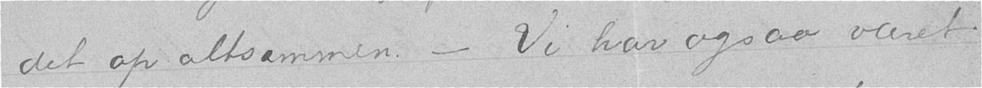det op altsammen. – Vi har ogsaa været
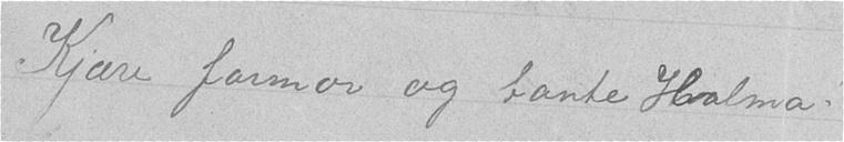Kjære farmor og tante Halma!
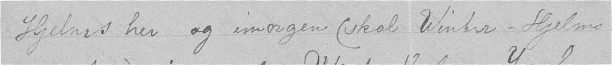Hjelms her og imorgen (skal Winter-Hjelm
